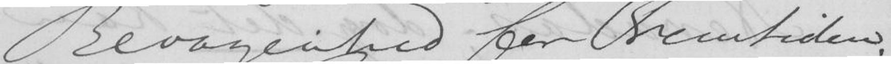Bevagenhed for Fremtiden.
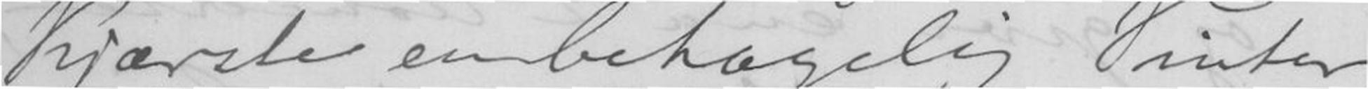Kjærste enbehagelig Vinter
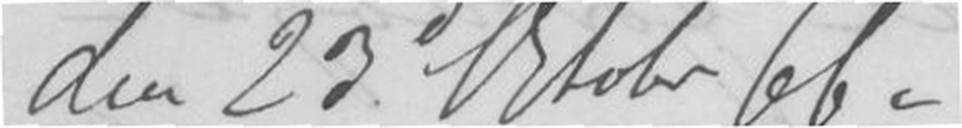den 23d Octobr. 66
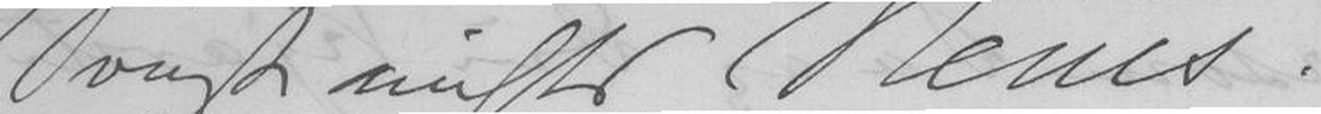Øvrigt nichts Neues.
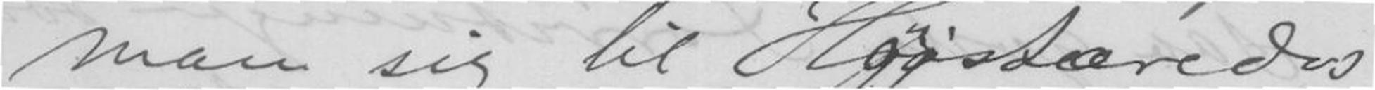man sig til Højstæredes
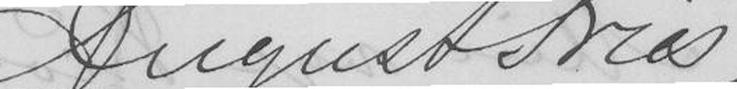August Fries
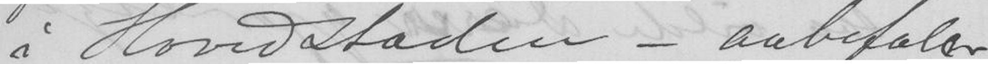i Hovedstaden - anbefaler
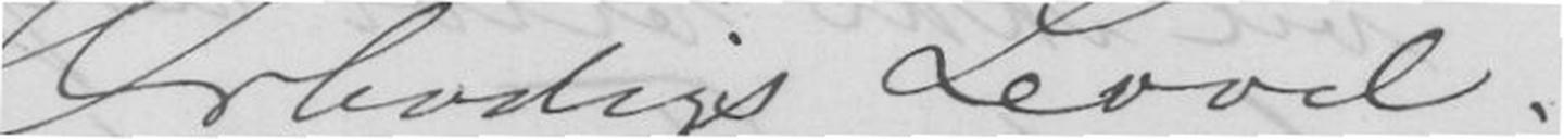Ærbødigs Levvel.
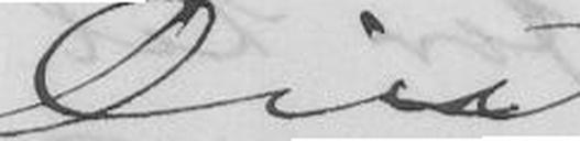Din
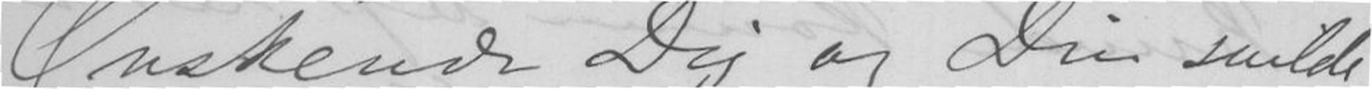Ønskende Dig og Din snilde
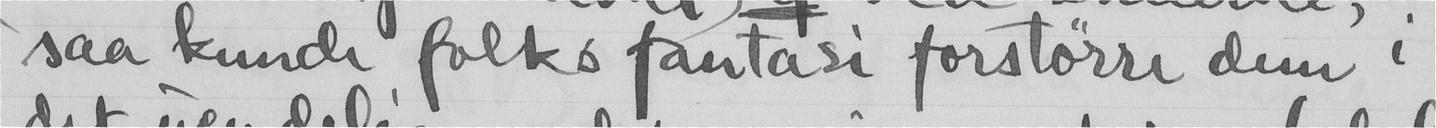saa kunde folks fantasi forstørre dem i
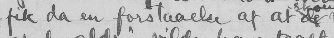fik da en forstaaelse af at de
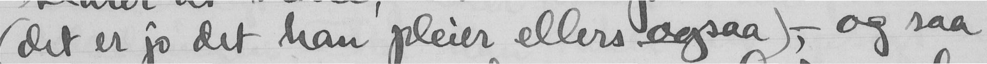det er jo det han pleier ellers ogsaa), - og saa
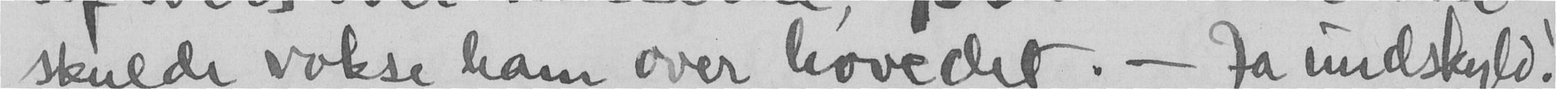skulde vokse ham over hovedet. - Ja undskyld!
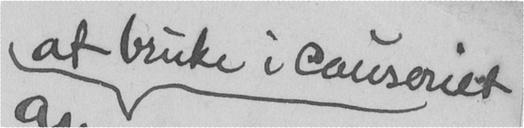at bruke i causeriet
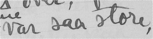var saa store,
